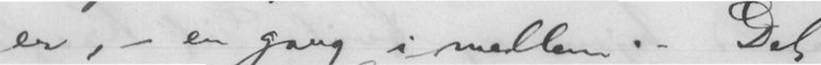er, - en gang imellem.- Det
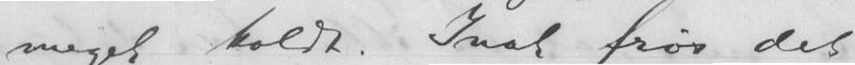meget koldt. Inat frøs det
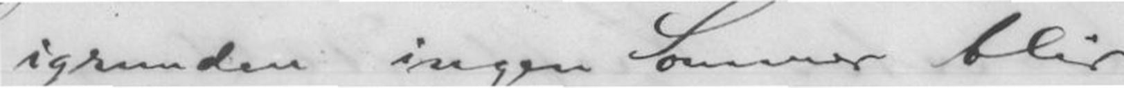igrunden ingen Sommer blir
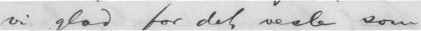vi glad for det vesle som
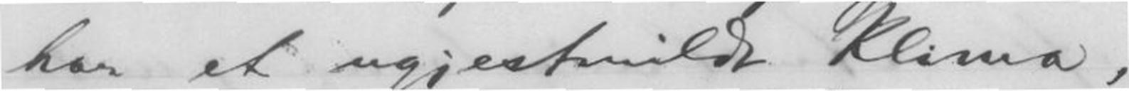har et ugjestmildt Klima,
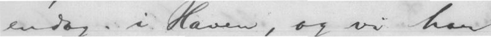endog i Haven, og vi har
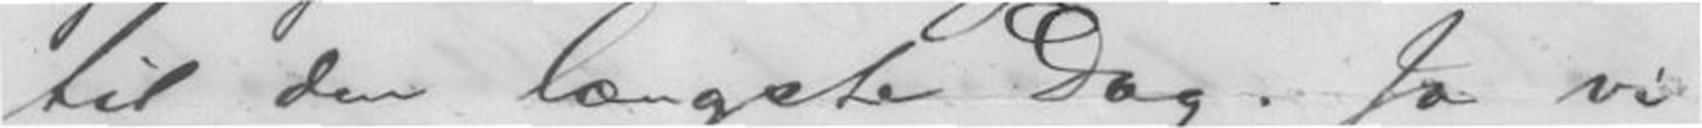til den længste Dag. Ja vi
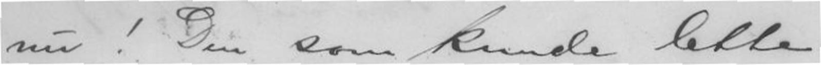nu! Den som kunde lette
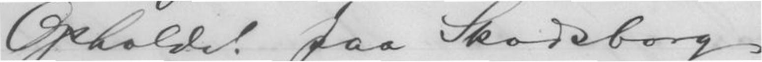Opholdet paa Skodsborg
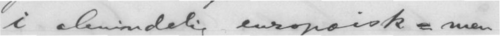i almindelig europæisk-men-
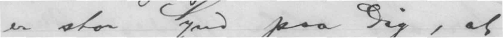er stor Synd paa Dig, at
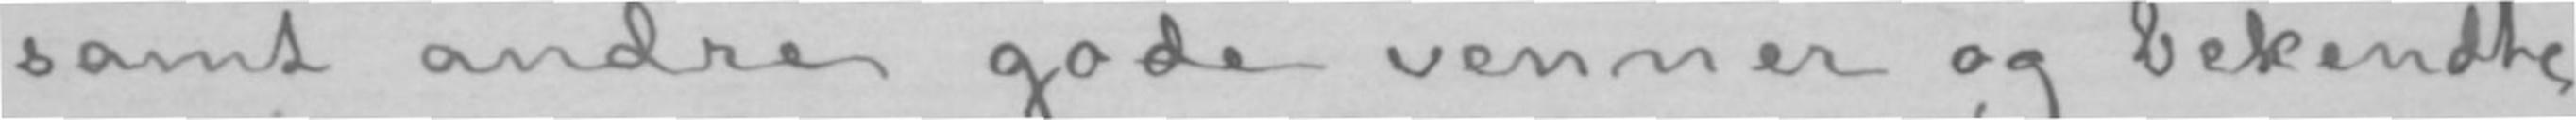samt andre gode venner og bekendte
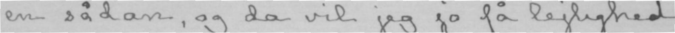en sådan, og da vil jeg jo få lejlighed
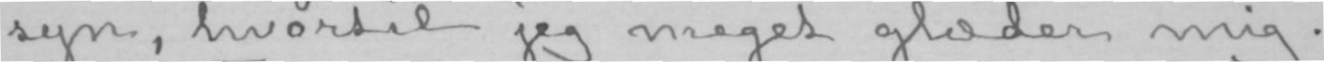syn, hvortil jeg meget glæder mig.
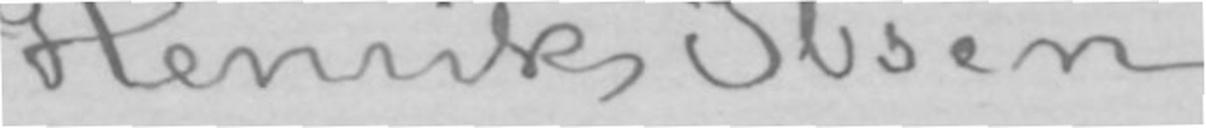Henrik Ibsen.
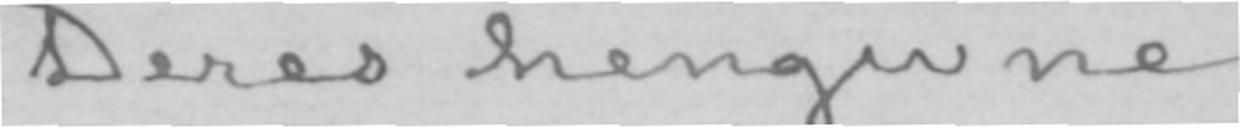Deres hengivne
